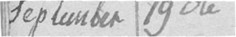September 19de
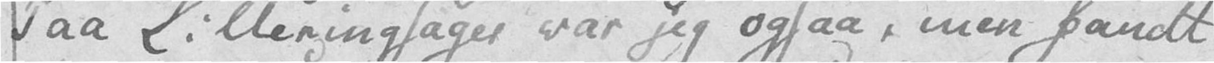Paa Lilleringsager var jeg ogsaa, men fandt
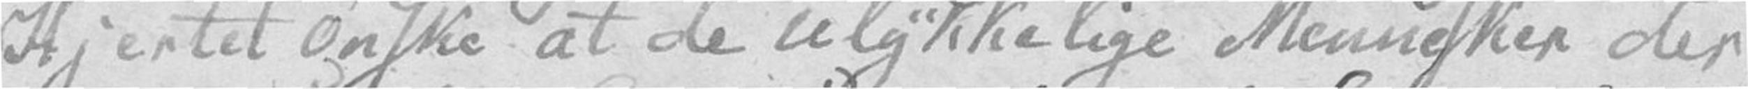Hjertet ønske at de Ulykkelige Mennesker der
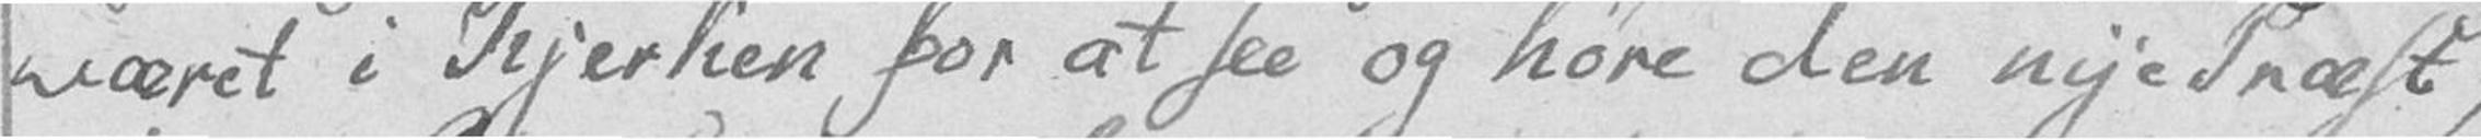været i Kjerken for at see og høre den nye Præst,
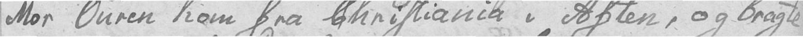Mor Ouren kom fra Christiania i Aften, og bragte
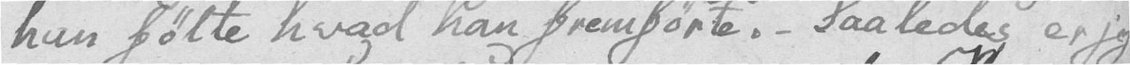han følte hvad han fremførte. – Saaledes er jeg
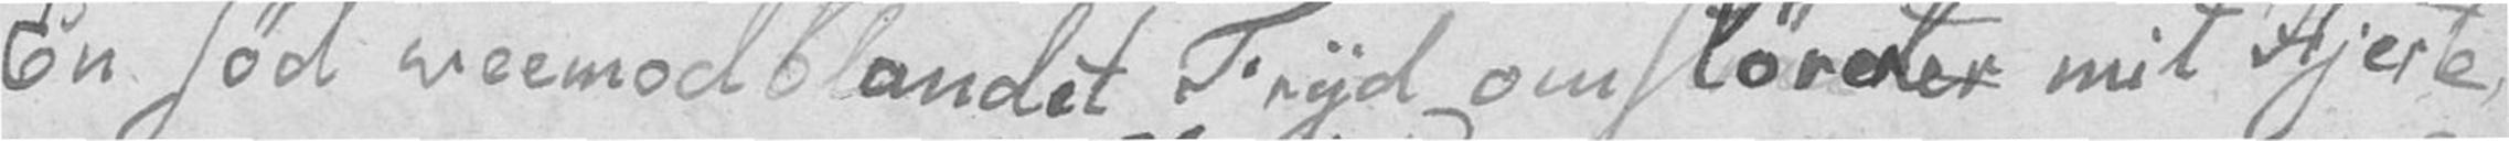En sød veemodblandet Fryd omslørerler mit Hjerte,
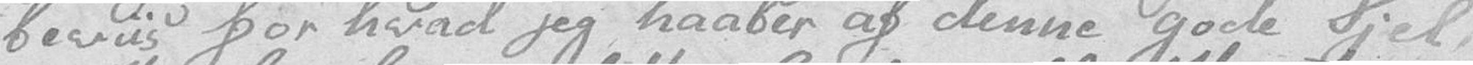beviis for hvad jeg haaber af denne gode Sjel,
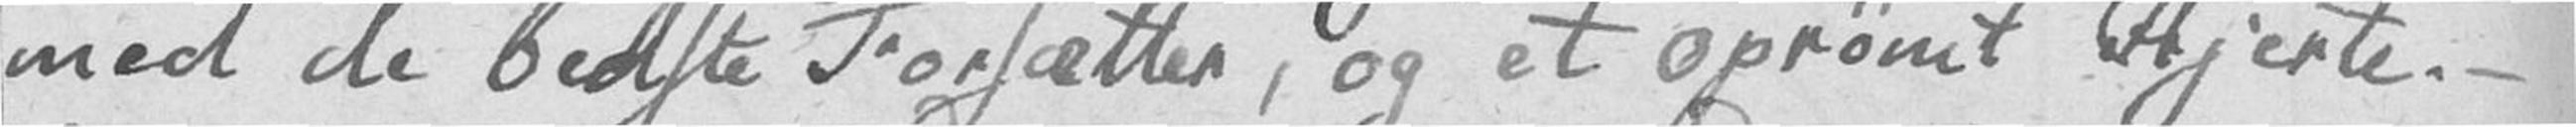med de bedste Forsætter, og et oprømt Hjerte. –
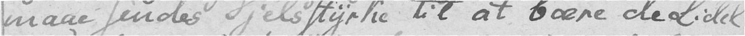maae sendes Sjelsstyrke til at bære de Lidel
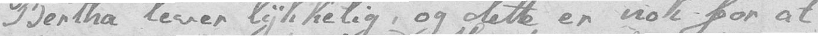Bertha lever lykkelig, og dette er nok for at
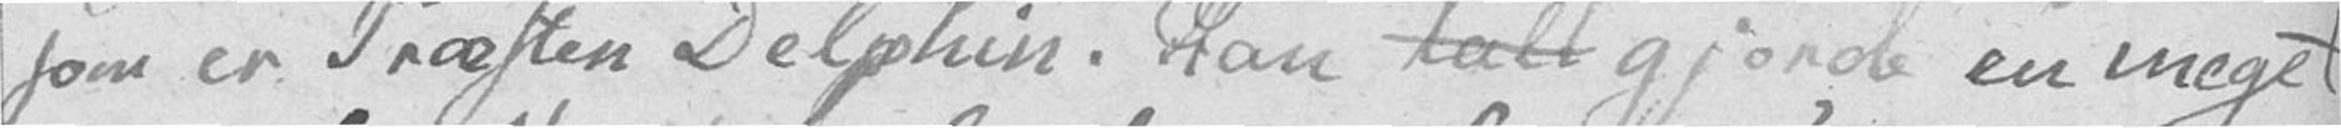som er Præsten Delphin. Han talt gjorde en meget
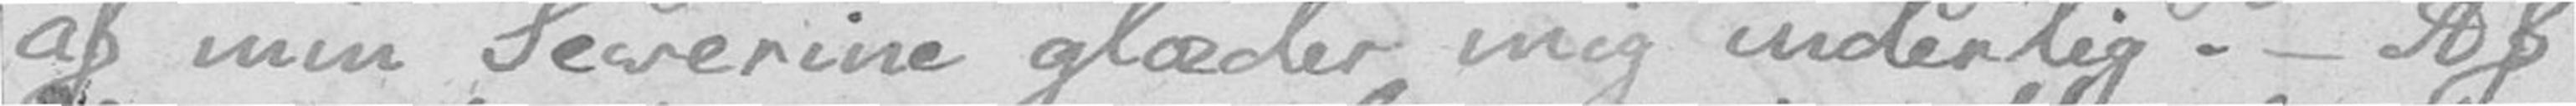af min Severine glæder mig inderlig. – Af
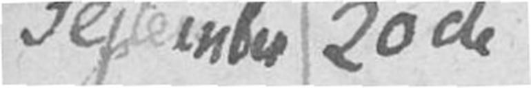September 20de
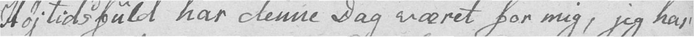Højtidsfuld har denne Dag været for mig, jeg har
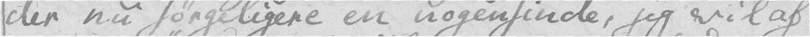der nu sørgeligere en nogensinde, jeg vil af
